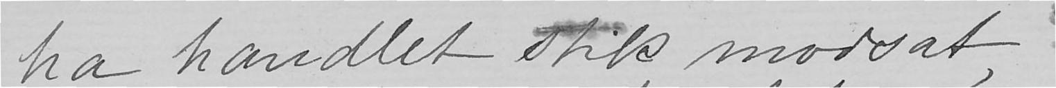ha handlet stik modsat,
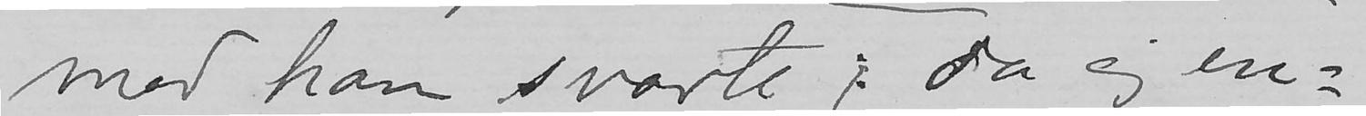med han svarte, da jeg en-
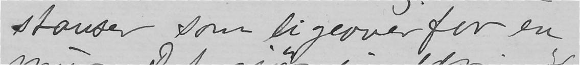stanser som ligeoverfor en
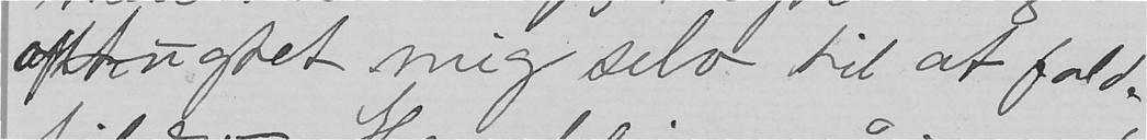optugtet mig selv til at falde
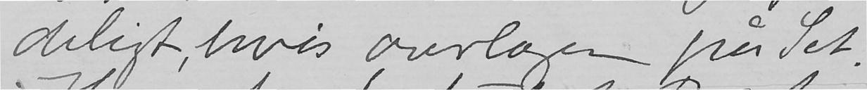deligt hvis overlægen på Sct.
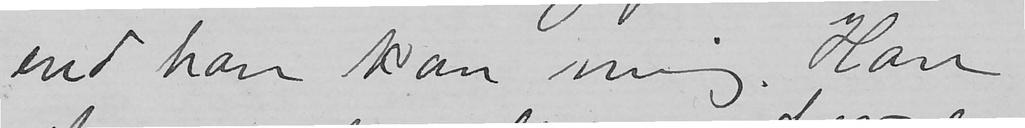end han kan mig. Han
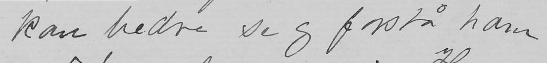kan bedre se og forstå ham
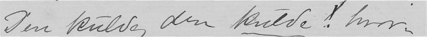Den kulde, den kulde! hvor-
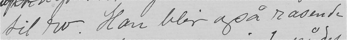til ro. Han blir også rasende
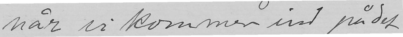når vi kommer ind på det.
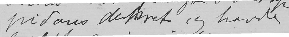pidans dekret og havde
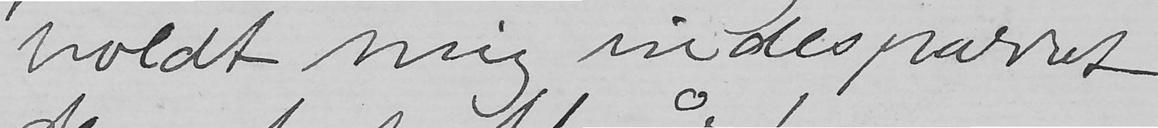holdt mig indespærret
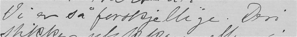Vi er så forskjellige. Deri
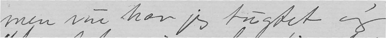men nu har jeg tugtet og
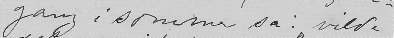gang i sommer sa: "vilde
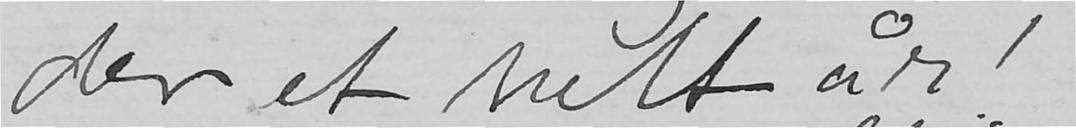der et helt år!
